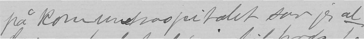på kommunehospitalet sov jeg al-
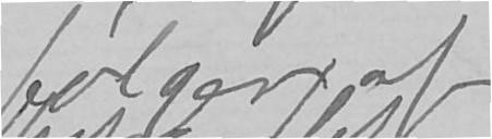følger af
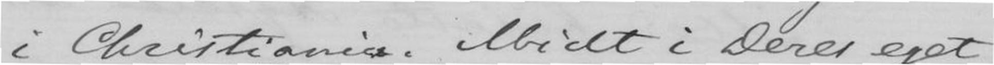i Christiania. Midt i Deres eget
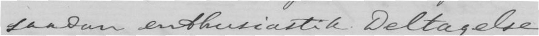saadan enthusiastik Deltagelse
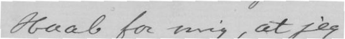Haab for mig, at jeg
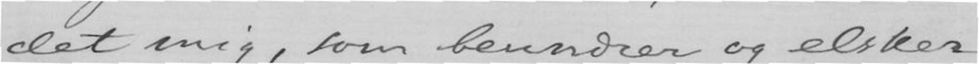det mig, som beundrer og elsker
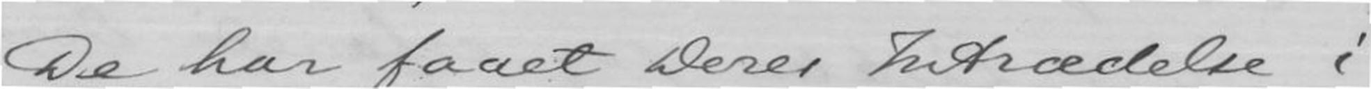De har faaet Deres Indtrædelse i
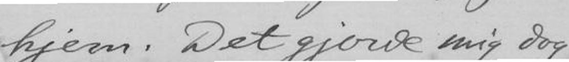hjem. Det gjorde mig dog
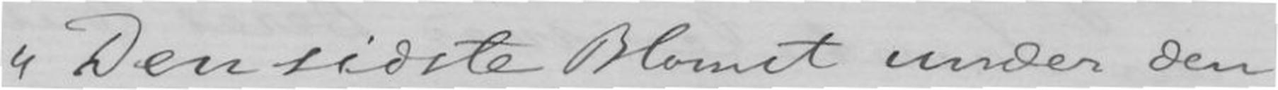Den sidste Blomst under den
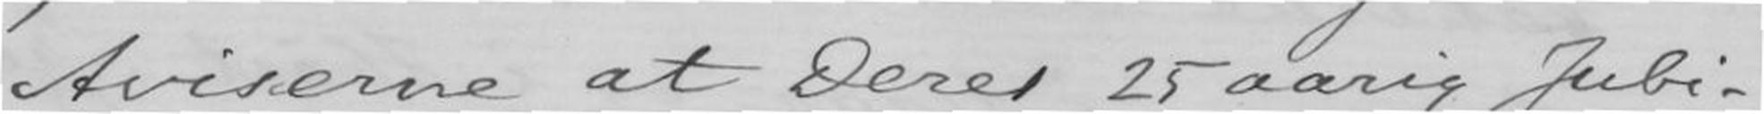Aviserne at Deres 25 aarig Jubi-
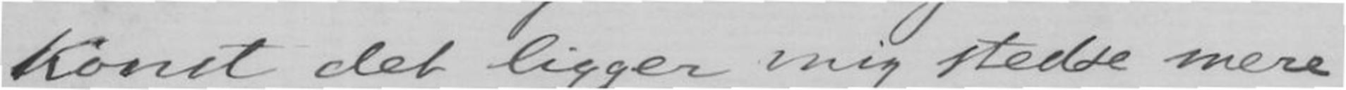Konst det ligger mig stedse mere
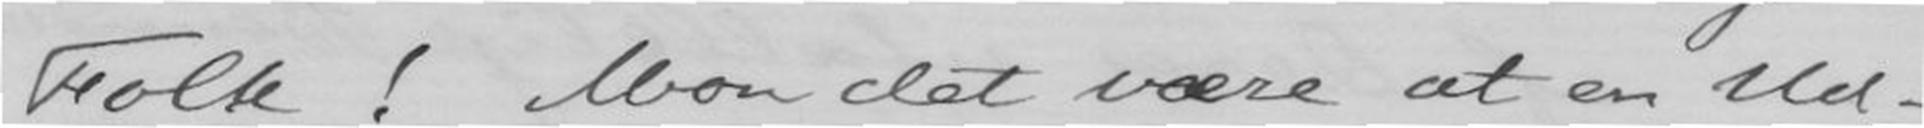Folk! Mon det være at en Ud-
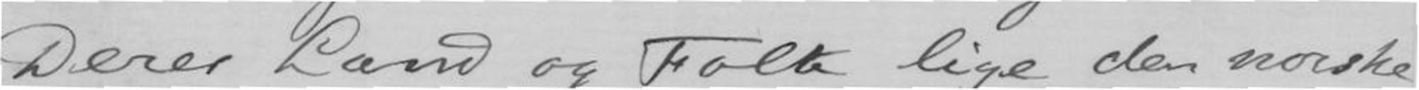Deres Land og Folk lige den norske
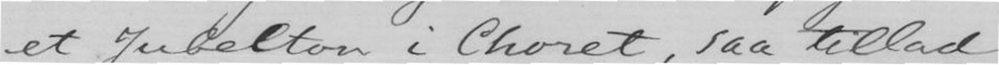et Jubelton i Choret, saa tillad
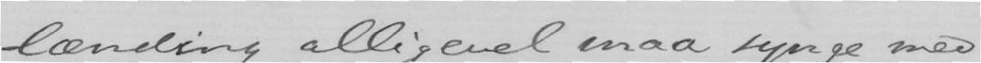lænding alligevel maa synge med
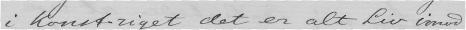i konst-riget det er alt Liv imod
